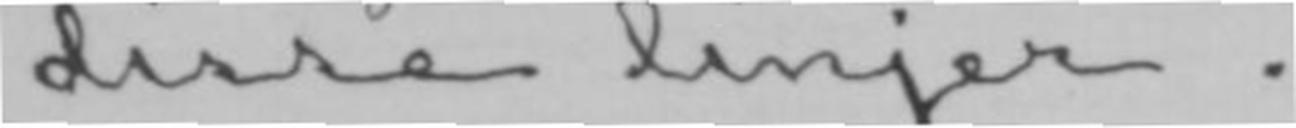disse linjer.
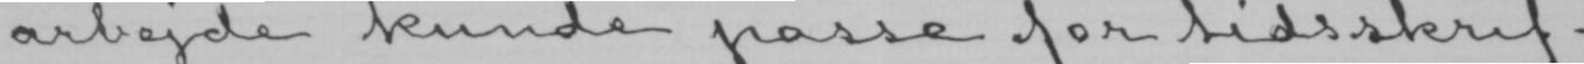arbejde kunde passe for tidsskrif-
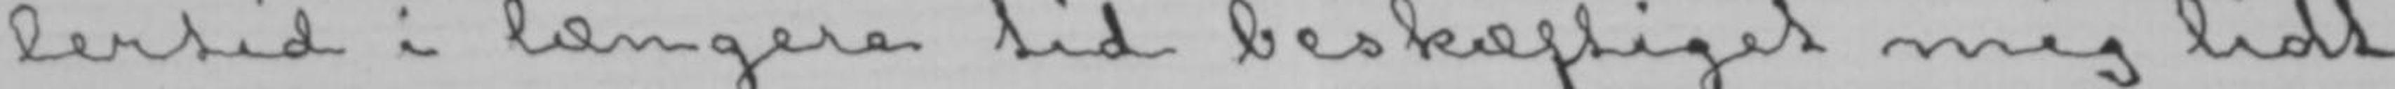lertid i længere tid beskæftiget mig lidt
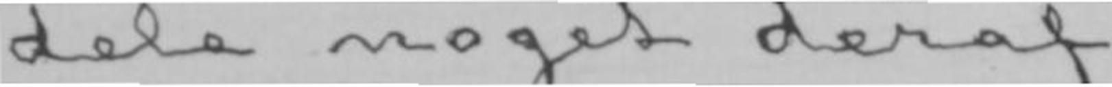dele noget deraf.
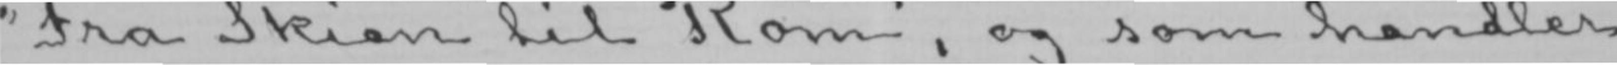«Fra Skien til Rom», og som handler
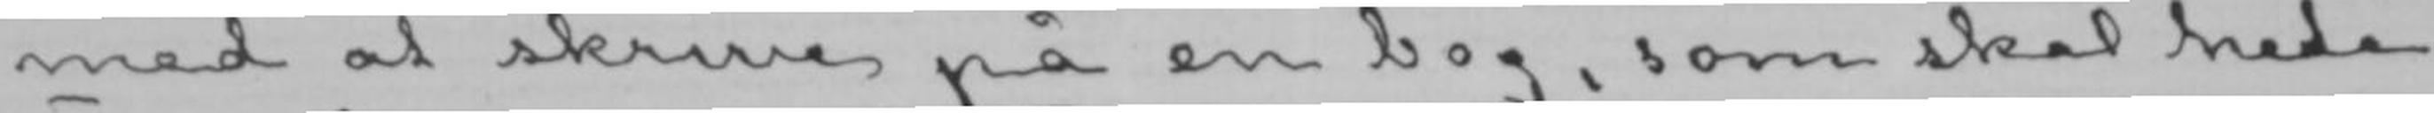med at skrive på en bog, som skal hede
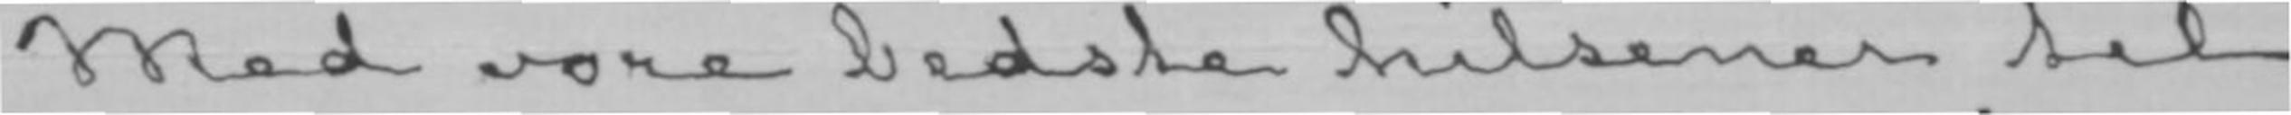Med vore bedste hilsener til
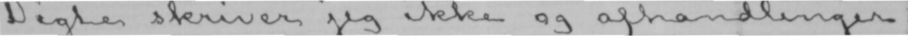Digte skriver jeg ikke og afhandlinger
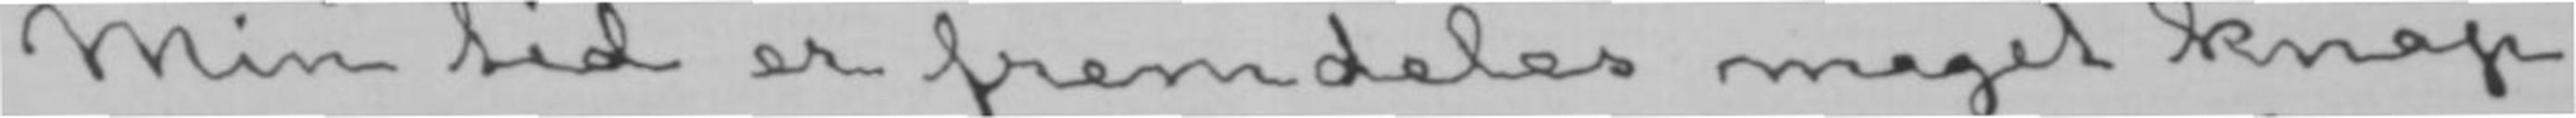Min tid er fremdeles meget knap
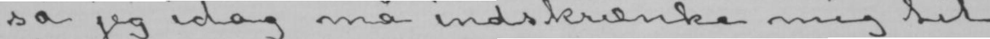så jeg idag må indskrænke mig til
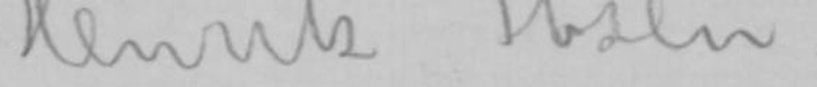Henrik Ibsen
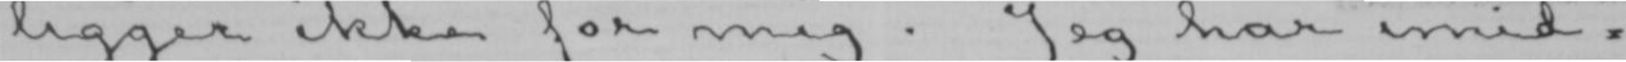ligger ikke for mig. Jeg har imid-
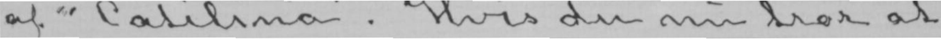af «Catilina». Hvis du nu tror at
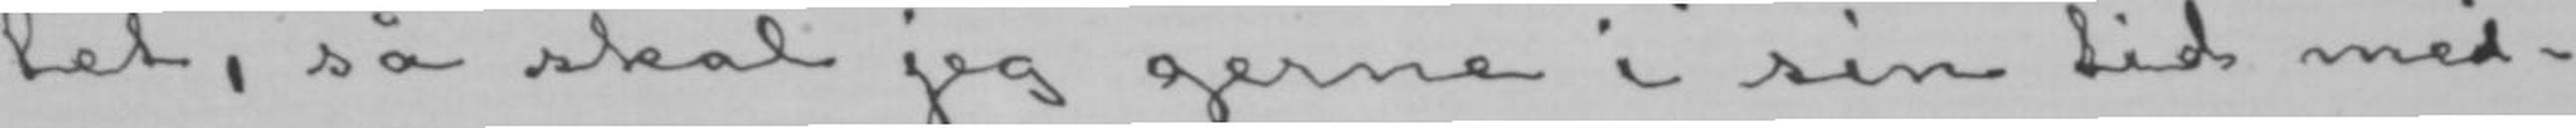tet, så skal jeg gerne i sin tid med-
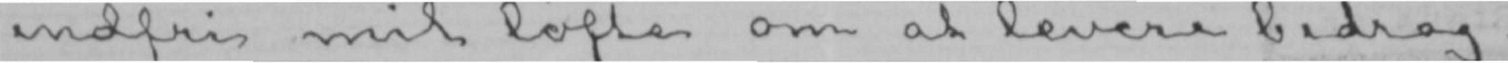indfri mit løfte om at levere bidrag.
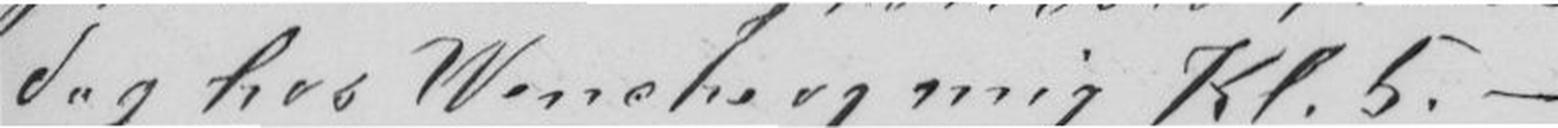dag hos Wenche og mig Kl. 5. -
